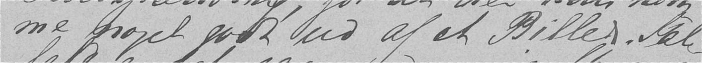me noget godt ud af et Billed. Ial-
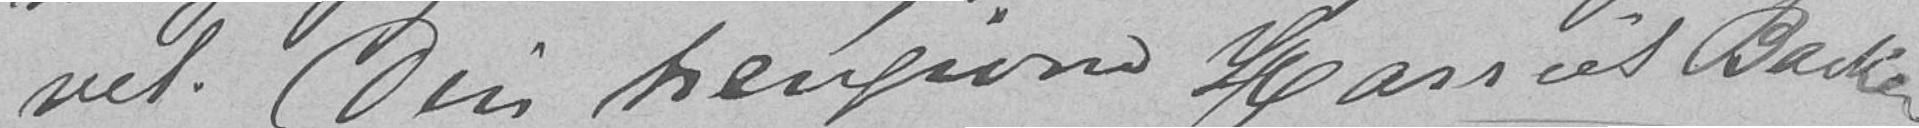vel. Din hengivne Harriet Backer
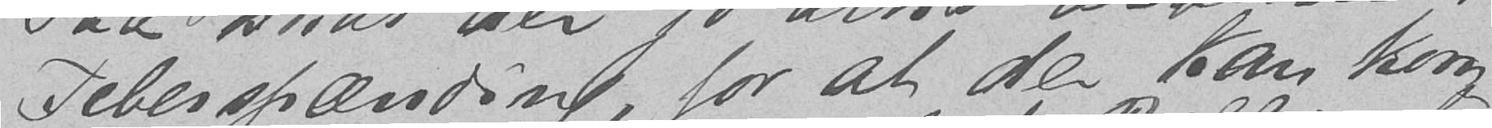Feberspænding, for at der kan kom-
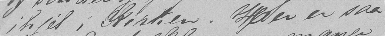ihjel i Kirken. Her er saa
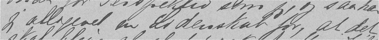jeg alligevel en Lidenskab for, at det
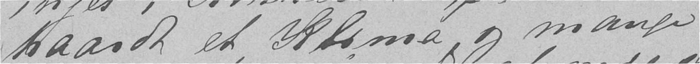baardt et Klima og mange
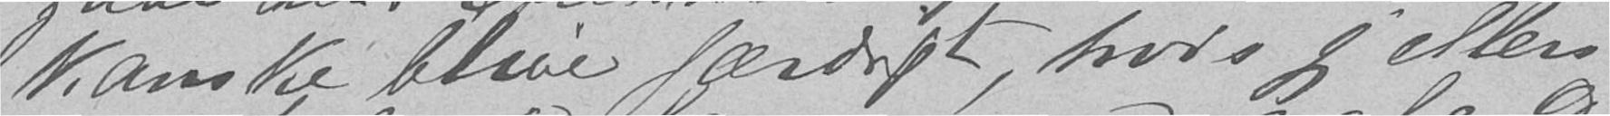kanske blive færdigt, hvis jeg ellers
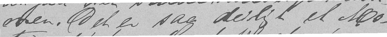men. Det er saa deiligt et Mo-
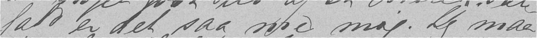fald er det saa med mig. Jeg maa
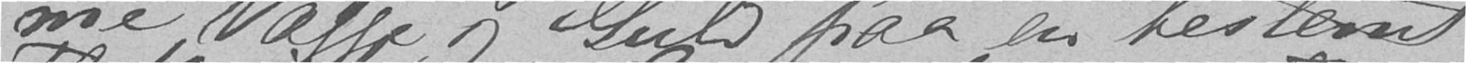me Vægge og Gulv paa en bestemt
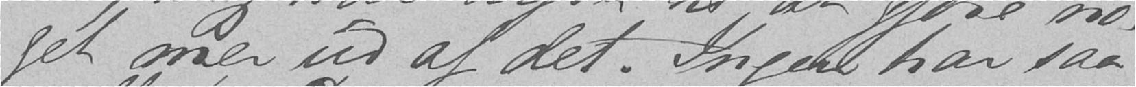get mer ud af det. Ingen har saa
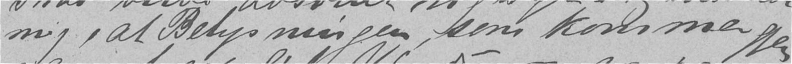mig, at Belysningen, som kommer gjen-
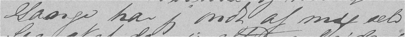Gange har jeg ondt af mig selv
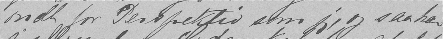ondt for Perspektiv som jeg, og saa har
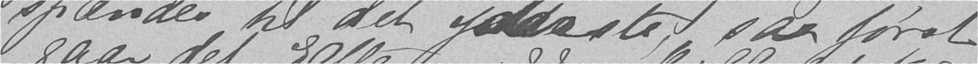spændes til det ydderste, saa først
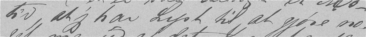tiv, at jeg har Lyst til at gjøre no-
